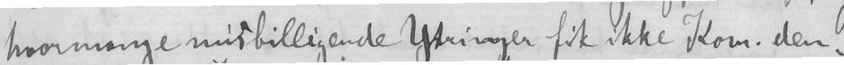hvormange misbilligende Ytringer fik ikke Kom. den
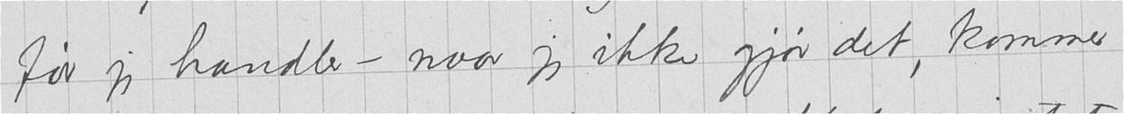før jeg handler - naar jeg ikke gjør det, kommer
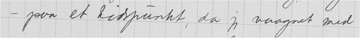– paa et tidspunkt, da jeg vaagnet med
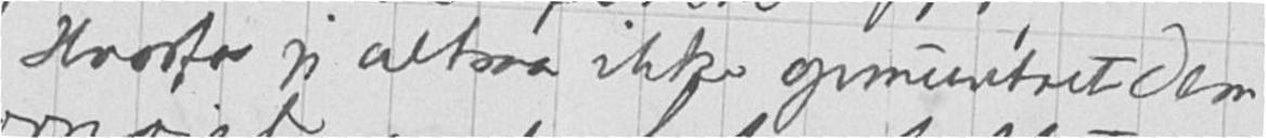Hvorfor jeg altsaa ikke opmuntret Dem
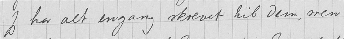Jeg har alt engang skrevet til Dem, men
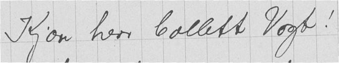Kjære herr Collett Vogt!
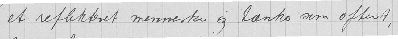et reflekteret menneske og tænker som oftest,
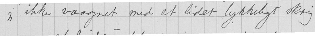jeg ikke vaagnet med et lidet lykkeligt skrig
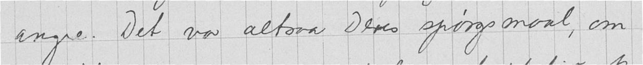angre. Det var altsaa Deres spørgsmaal, om
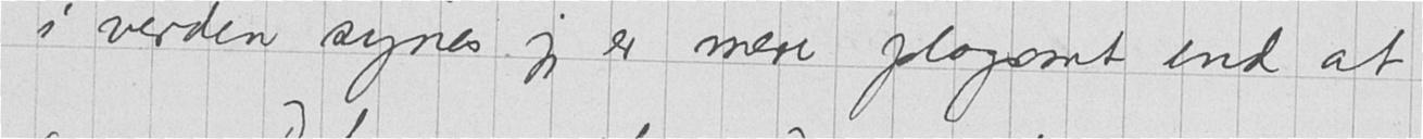i verden synes jeg er mere plagsomt end at
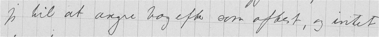jeg til at angre bagefter som oftest, og intet
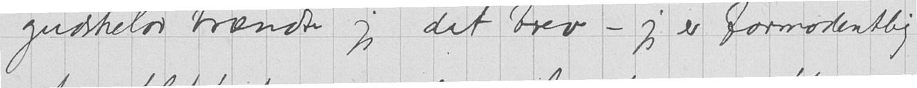gudskelov brændte jeg det brev – jeg er formodentlig
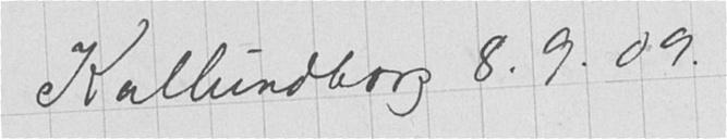Kallundborg 8.9.09.
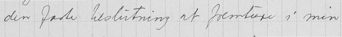den faste beslutning at fremture i min
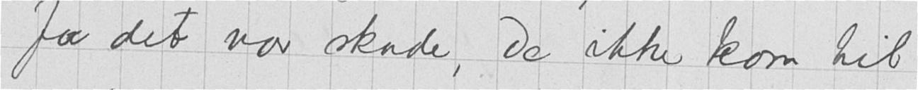Ja det var skade, De ikke kom til
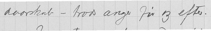daarskab – trods anger før og efter.
